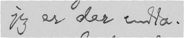jeg er der endda.
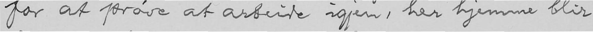for at prøve at arbeide igjen, her hjemme blir
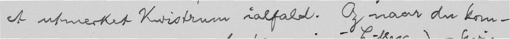et utmerket Kvistrum ialfald. Og naar du kom-
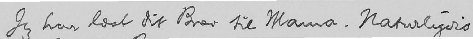Jeg har læst dit Brev til Mama. Naturligvis
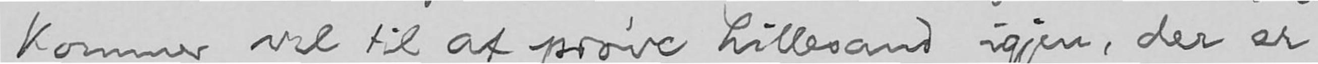kommer vel til at prøve Lillesand igjen, der er
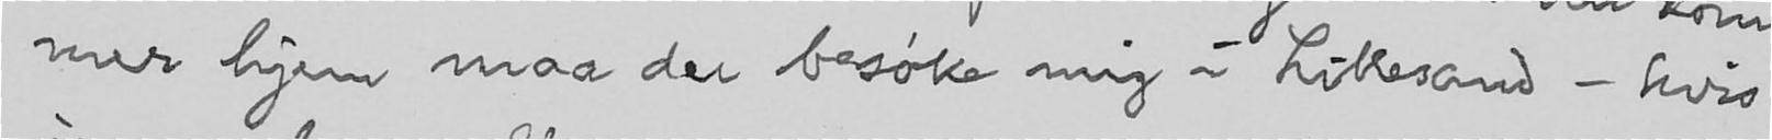mer hjem maa du besøke mig i Lillesand - hvis
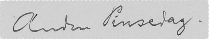Anden Pinsedag.
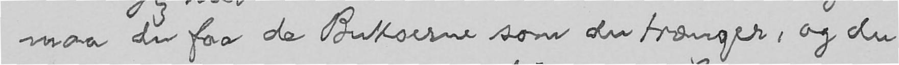maa du faa de Bukserne som du trænger, og du
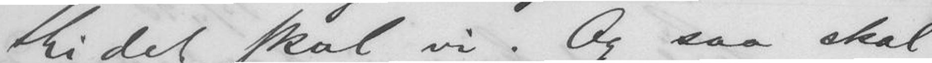thi det skal vi. Og saa skal
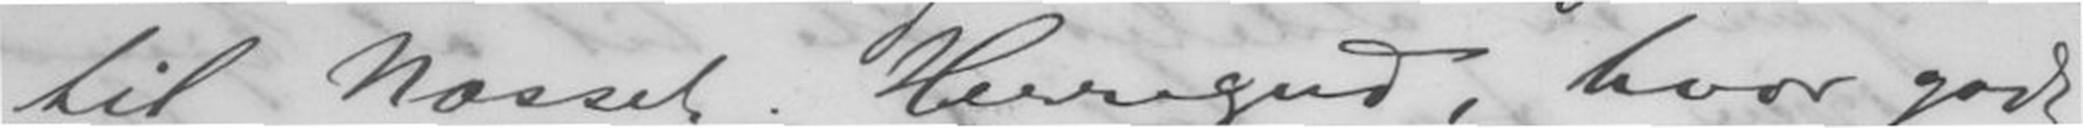til Næsset. Herregud, hvor godt
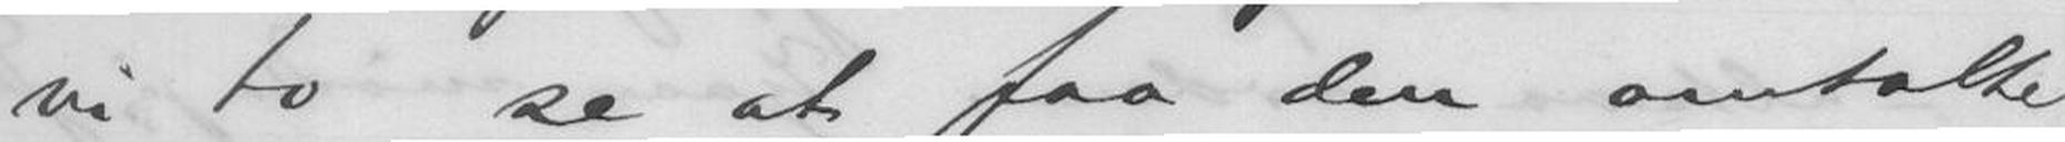vi to se at faa den omtalte
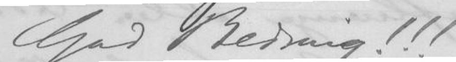God Bedring!!!
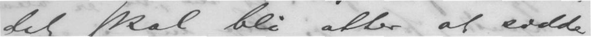det skal bli atter at sidde
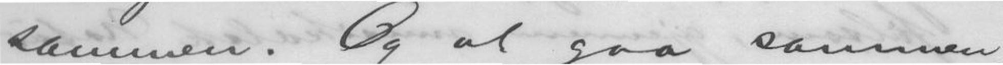sammen. Og at gaa sammen,
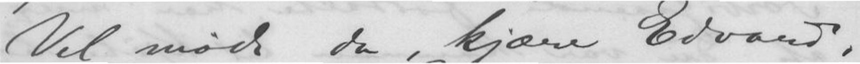Vel mødt da, kjære Edvard,
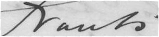Frants
